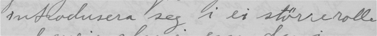introdusera seg i ei större rolle
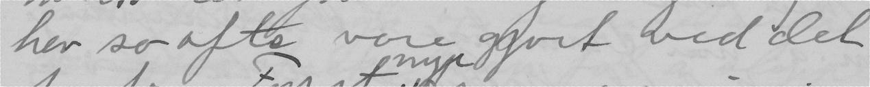hev so ofte vore gjort ved det
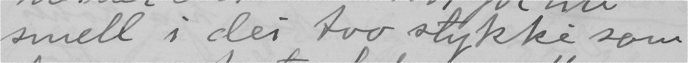smell i dei tvo stykki som
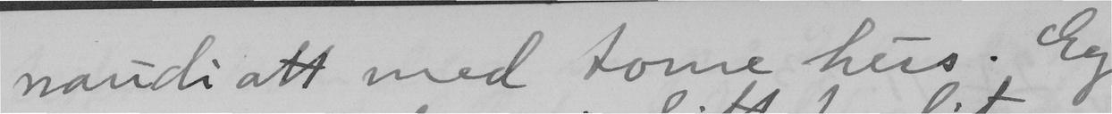naudi att med tome hus. Eg
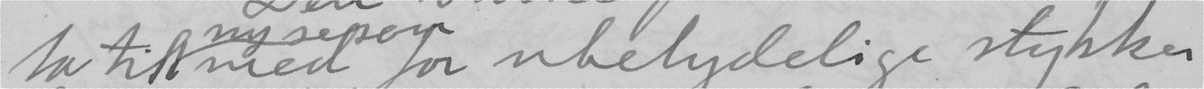ta til ny seson med for ubetydelige stykker
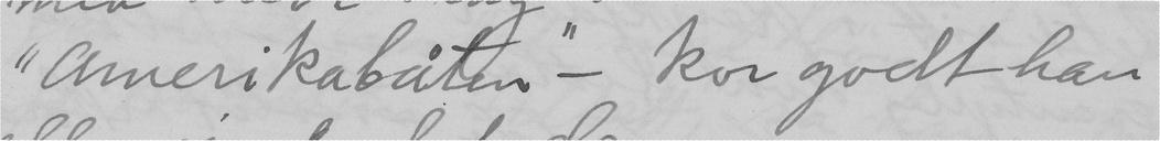"Amerikabåten" – kor godt han
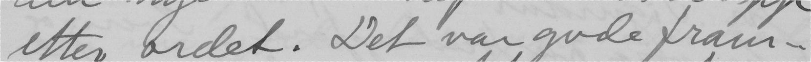etter ordet. Det var gode fram-
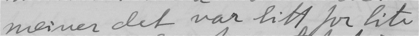meiner det var litt for lite
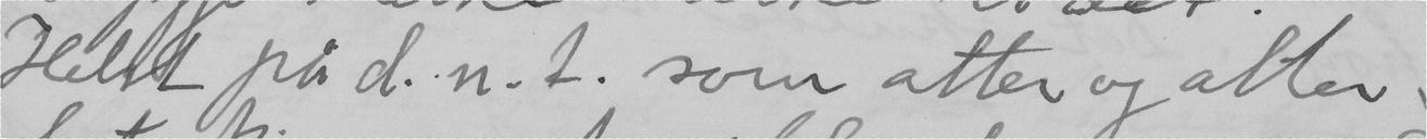Helst på d.n.t. som atter og atter.
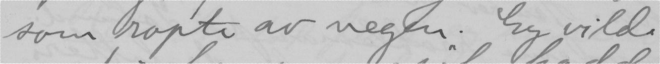som ropte av vegen. En vilde
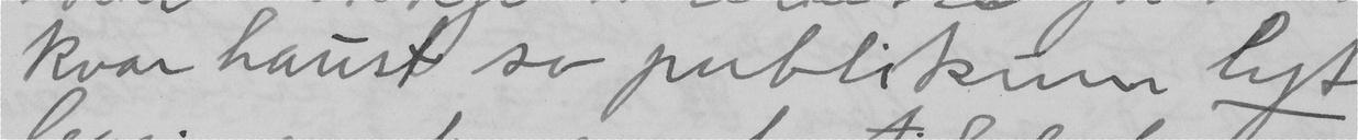kvar haust so publikum lyt
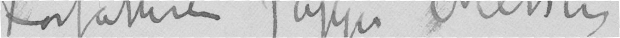Forfatteren Jappe Nilssen
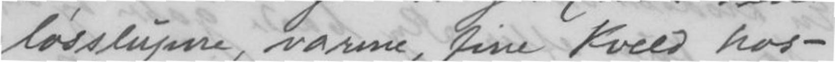løsslupne, varme, fine Kveld hos-
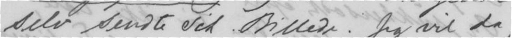selv sendte sit Billede. Jeg vil da,
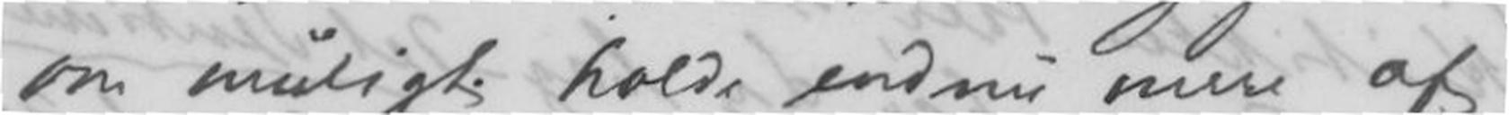om muligt holde endnu mere af
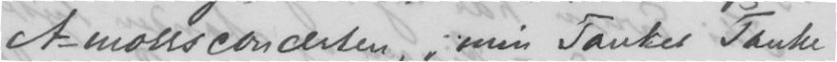A-mollsconcerten, min Tanker Tænke,
